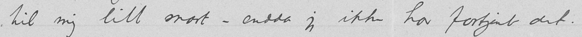til mig litt snart - endda jeg ikke har fortjent det.
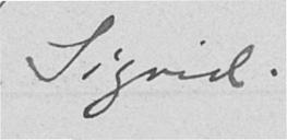Sigrid.
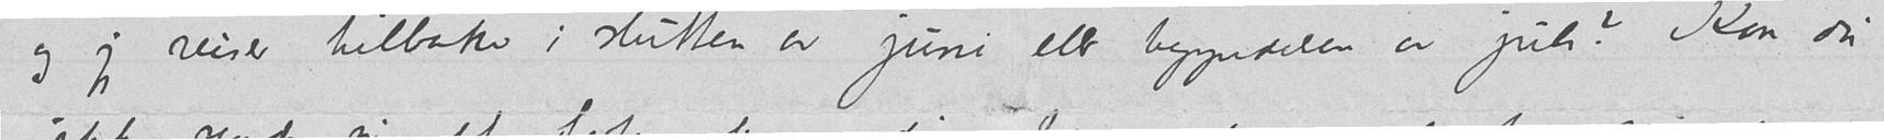og jeg reiser tilbake i slutten er juni ell begyndelsen av juli? Kan du
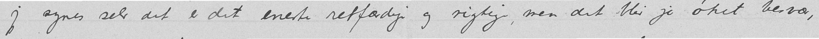jeg synes selv det er det eneste retfædige og rigtige, men det blir jo øket besvær,
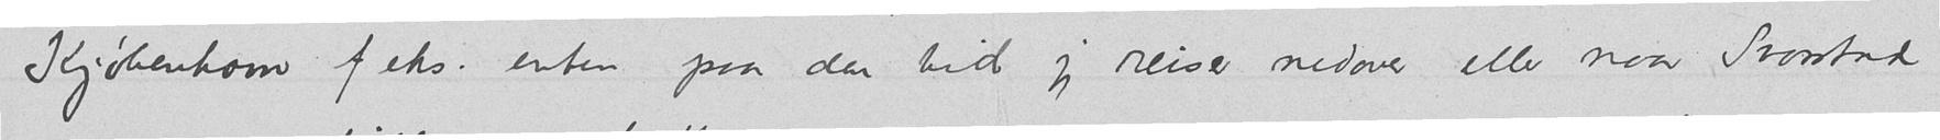Kjøbenhavn f.eks. enten paa den tid jeg reiser nedover eller naar Svarstad
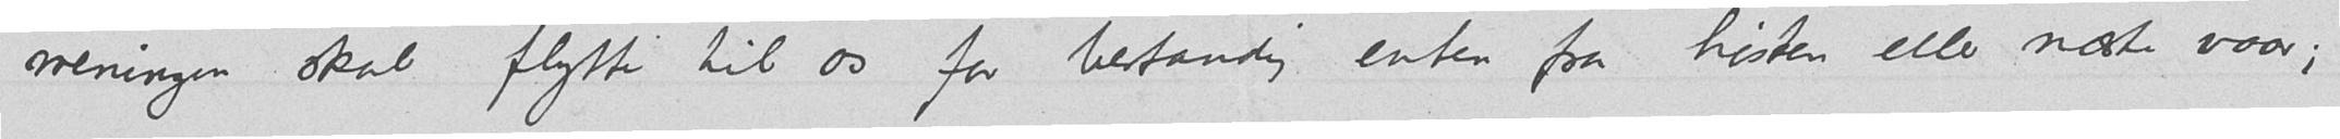meningen skal flytte til os for bestandig enten fra høsten eller næste vaar;
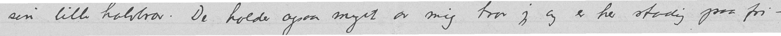sin lille halvbror. De holder ogsaa meget av mig tror jeg og er her stadig paa fri-
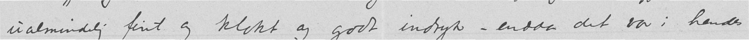ualmindelig fint og klokt og godt indryk - endda det var i hendes
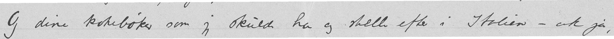Og dine kokebøker som jeg skulde ha og stelle efter i Italien - ak ja,
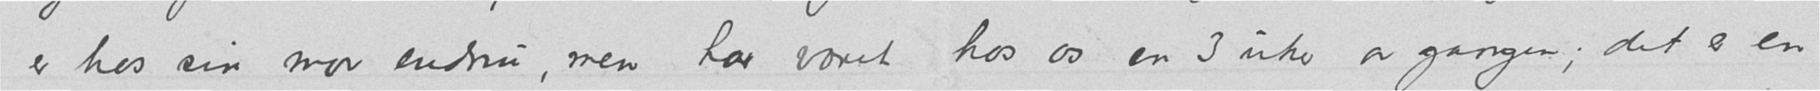er hos sin mor endnu, men har været hos os en 3 uker av gangen, det er en
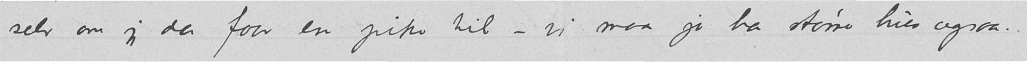selv om jeg da faar en pike til - vi maa jo ha større hus ogsaa.
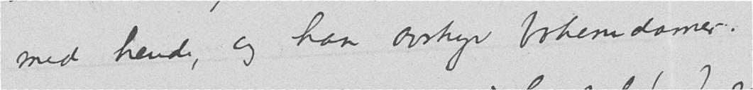med hende, og han avskyr bohemdamer.
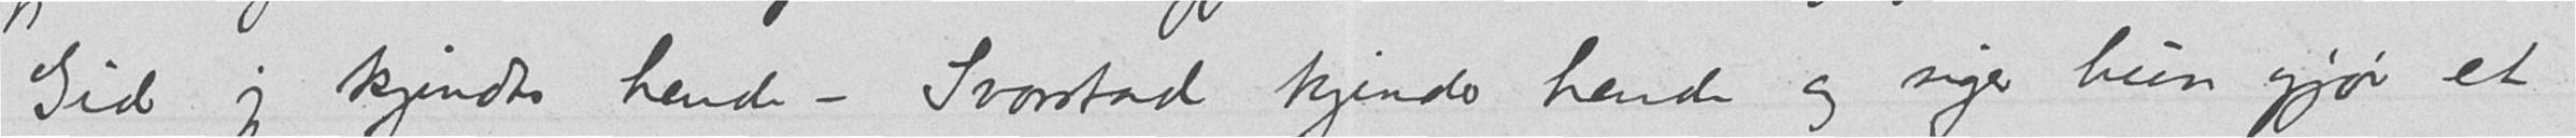Gid jeg kjendte hende - Svarstad kjender hende og siger hun gjør et
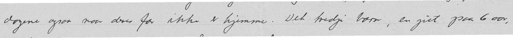dagene ogsaa naar deres far ikke er hjemme. Det tredje barn, en gut paa 6 aar,
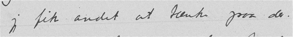jeg fik andet at tænke paa da.
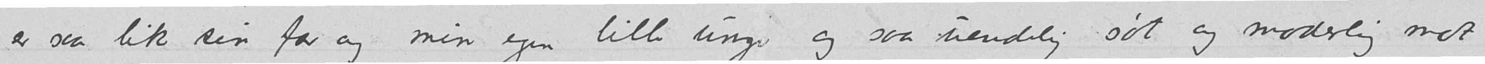er saa lik sin far og min egen lille unge og saa uendelig søt og moderlig mot
